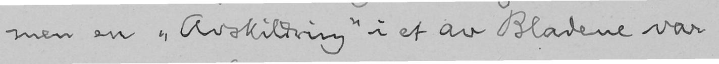men en "Avskildring" i et av Bladene var
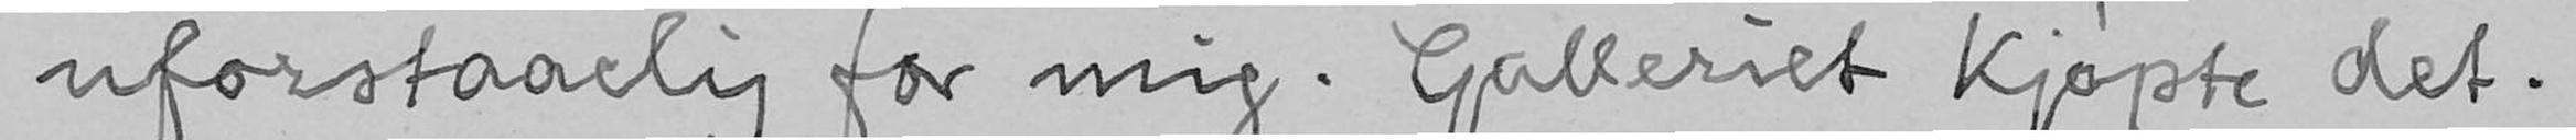uforstaaelig for mig. Galleriet kjøpte det.
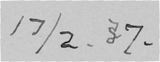17/2. 37.
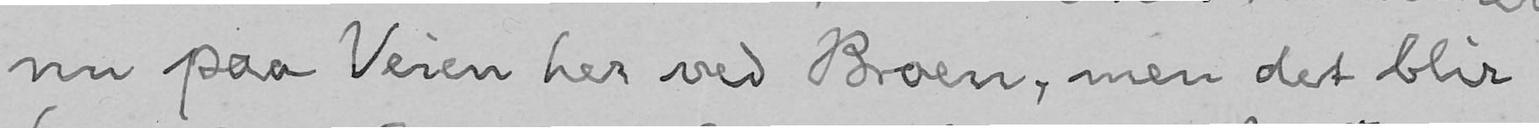nu paa Veien her ved Broen, men det blir
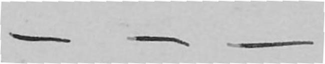- - -
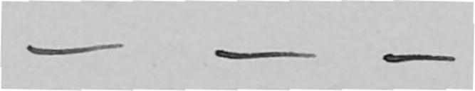- - -
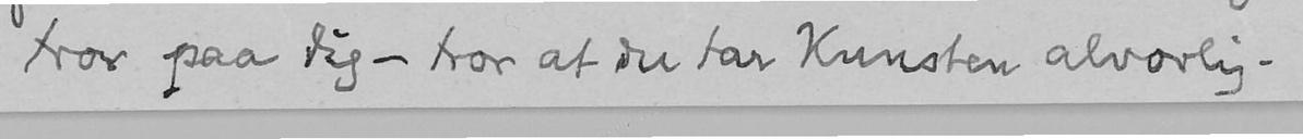tror paa dig - tror at du tar Kunsten alvorlig.
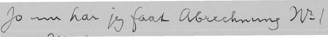Jo nu har jeg faat Abrechnung Nr 1
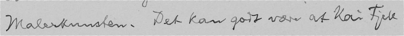Malerkunsten. Det kan godt være at Kai Fjell
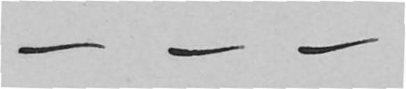- - -
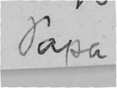Papa
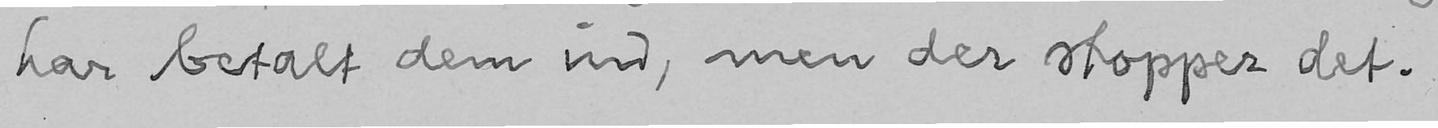har betalt dem ind, men der stopper det.
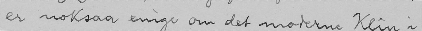er noksaa enige om det moderne Klin i
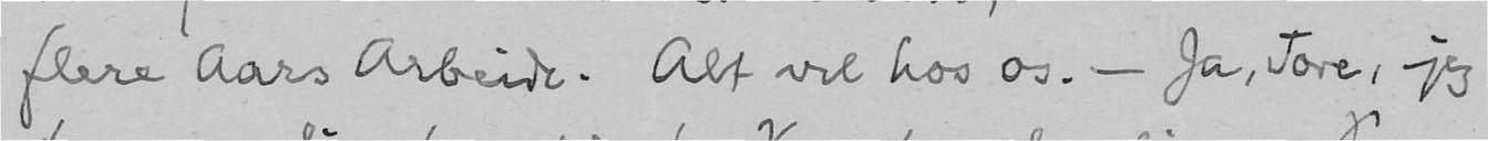flere Aars Arbeide. Alt vel hos os. - Ja, Tore, jeg
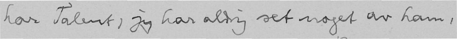har Talent, jeg har aldrig set noget av ham,
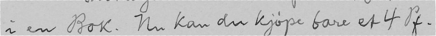i en Bok. Nu kan du kjøpe bare et 4 Pf.
